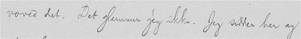voved det. Det glemmer jeg ikke. Jeg sitter her og
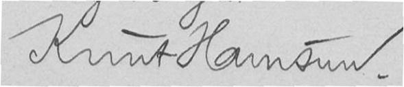Knut Hamsun.
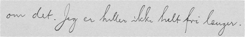om det. Jeg er heller ikke helt fri længer.
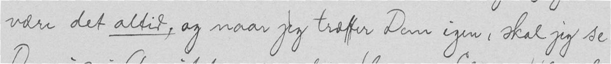være det altid, og naar jeg træffer Dem igen, skal jeg se
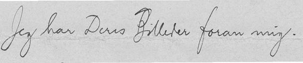Jeg har Deres Billeder foran mig.
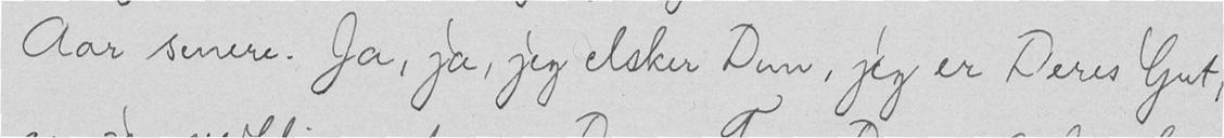Aar senere. Ja, ja, jeg elsker Dem, jeg er Deres Gut,
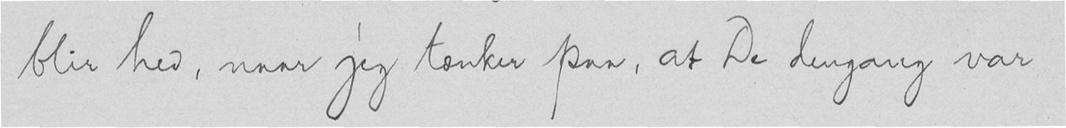blir hed, naar jeg tænker paa, at De dengang var
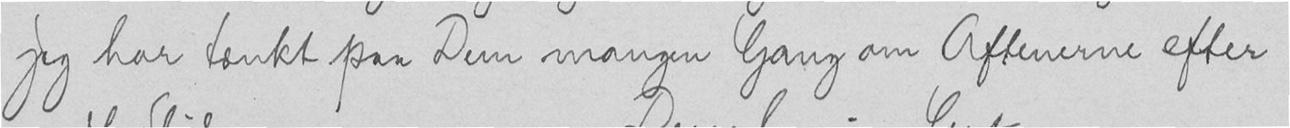jeg har tænkt paa Dem mangen Gang om Aftenerne efter
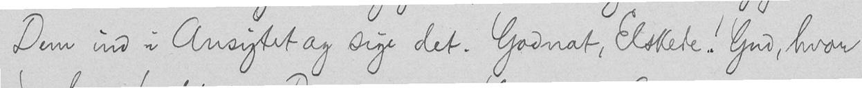Dem ind i Ansigtet og sige det. Godnat, Elskede! Gud, hvor
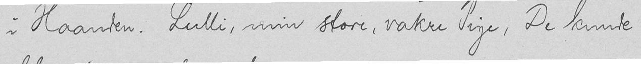i Haanden. Lulli, min store, vakre Pige, De kunde
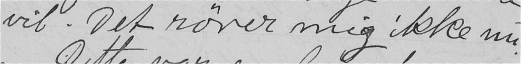vil. Det rører mig ikke nu
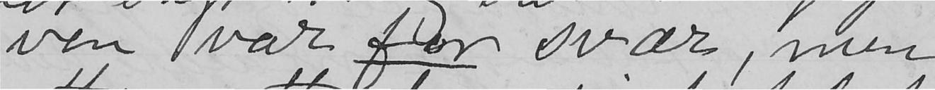ven var for svær, men
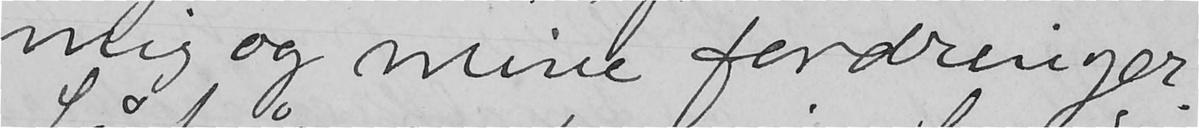mig og mine fordringer.
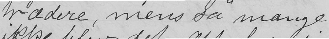trædere, mens så mange
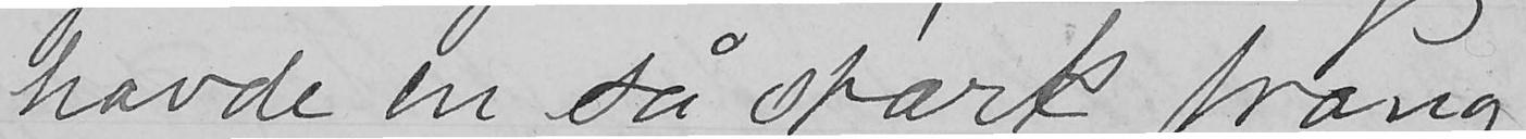havde en så stærk trang
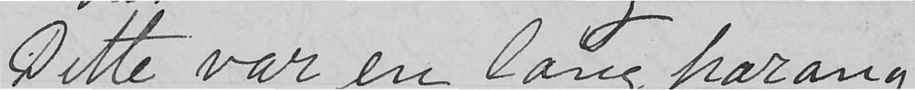Dette var en lang harang
